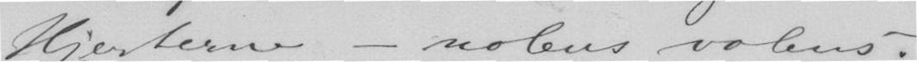Hjerterne - nolens volens.
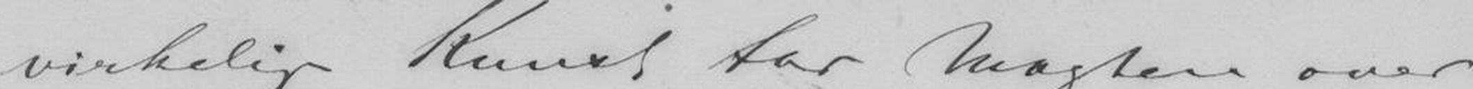virkelig Kunst tar Magten over
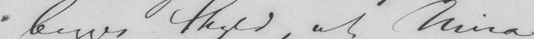begges Skyld, at Nina
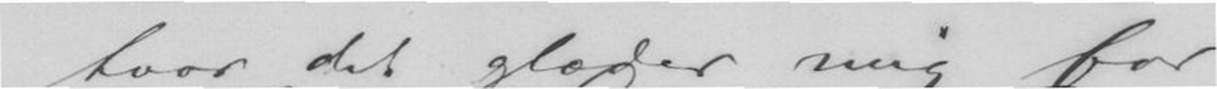hvor det glæder mig for
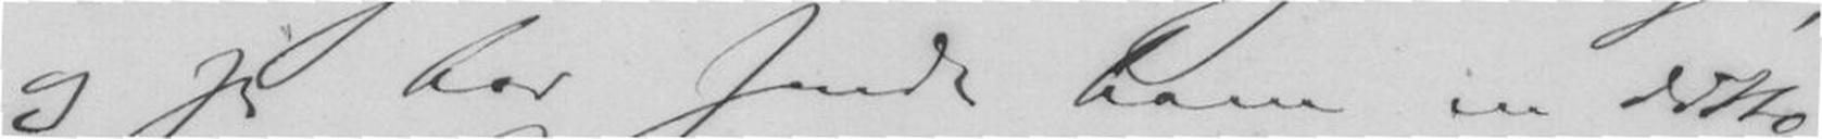og jeg har sendt ham en ditto
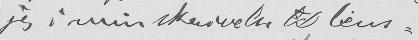jeg i min skrivelse til lens-
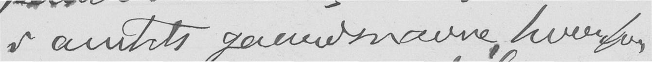i amtets gaardsnavne, hvorfor
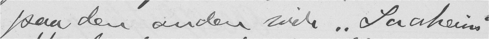paa den anden side "Saaheim"
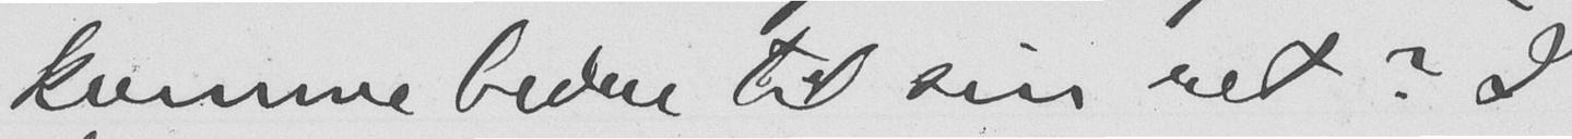komme bedre til sin ret? I
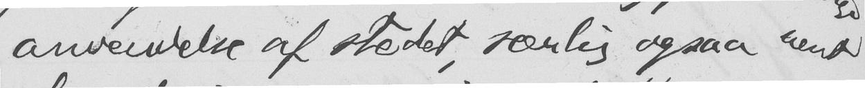anvendelse af stedet, særlig ogsaa rent
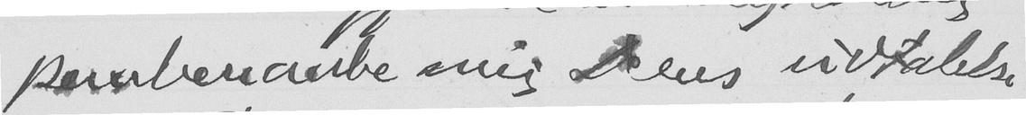paaberaabe mig Deres udtalelse
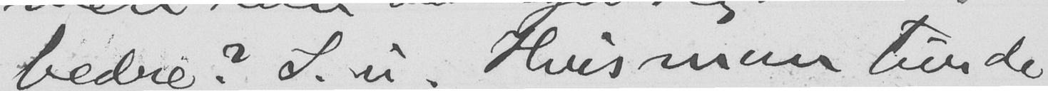bedre? S.u. Hvis man turde
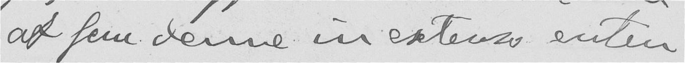at faa denne in extenso enten
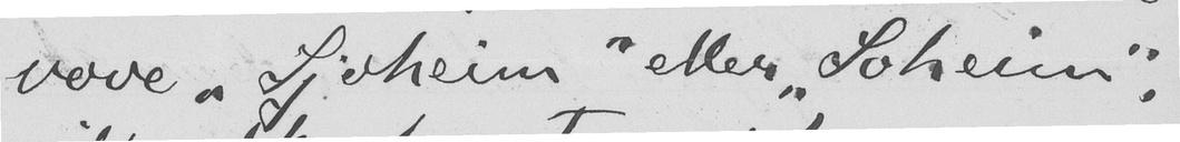vove "Sjoheim" eller "Soheim",
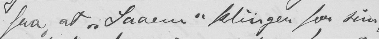fra, at "Saaem" klinger for sim-
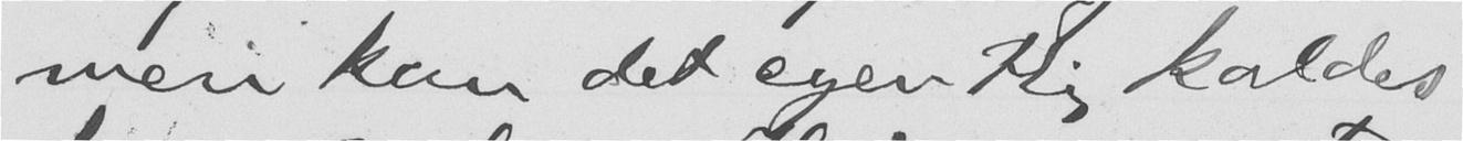men kan det egentlig kaldes
